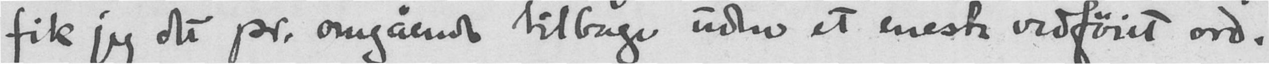fik jeg det pr. omgående tilbage uden et eneste vedføiet ord.
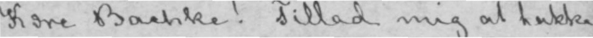Kære Bachke! Tillad mig at takke
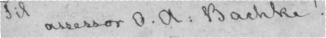Til assessor O. A: Bachke!
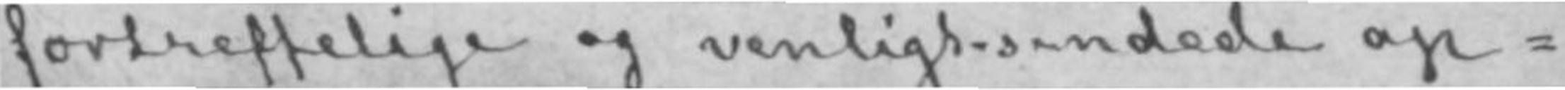fortreffelige og venligt-sindede op-
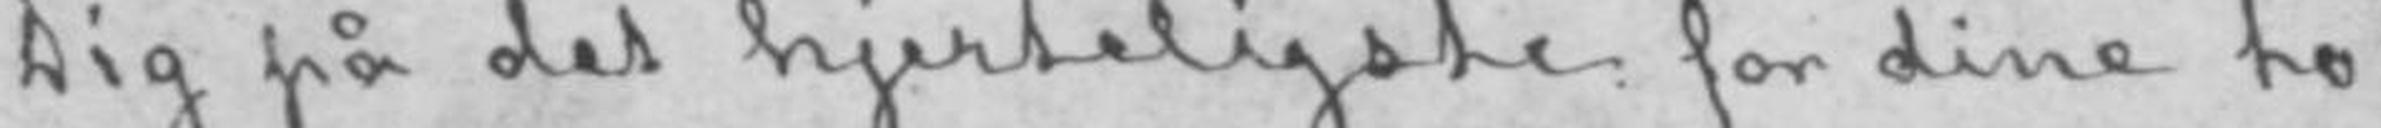Dig på det hjerteligste for dine to
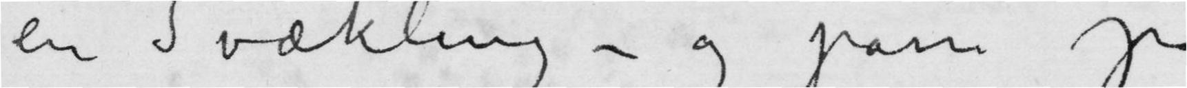en Svækling – og passe på
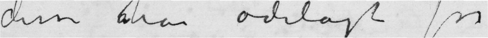disse har ødelagt for
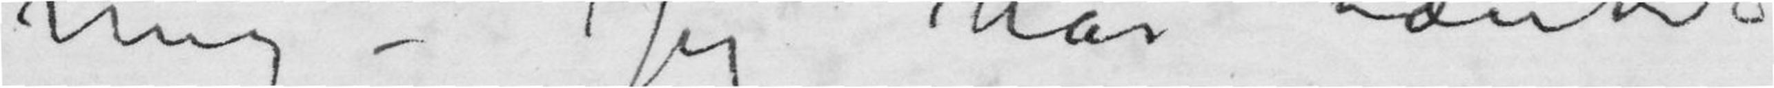mig – Jeg har tænkt
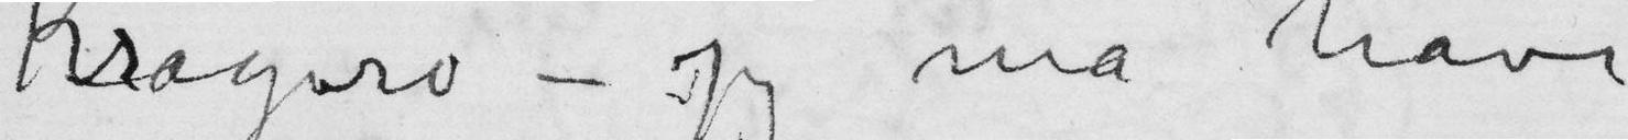Kragerø – Jeg må have
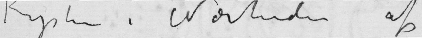Kysten i Nærheden af
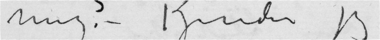mig? – Kjender jeg
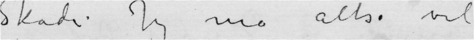Skade. Jeg må altså vel
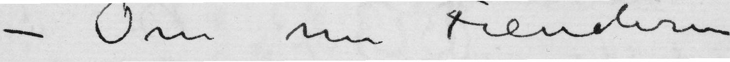– Om nu Fienderne
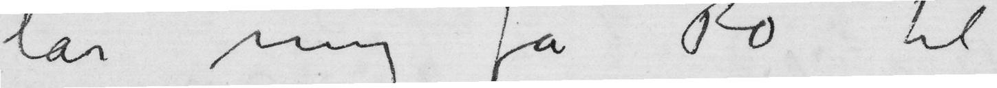lar mig få Ro til
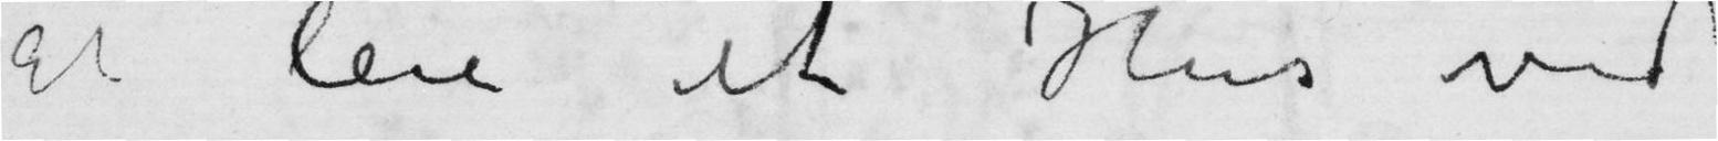at leie et Hus ved
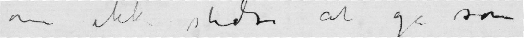om ikke stedse at gå som
